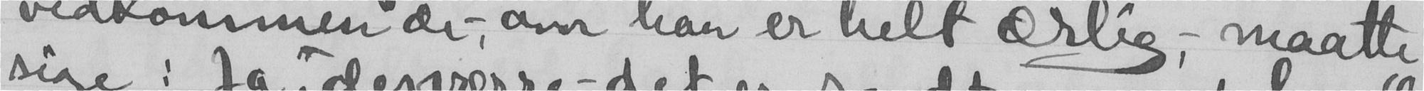vedkommende -, om han er helt ærlig, - maatte
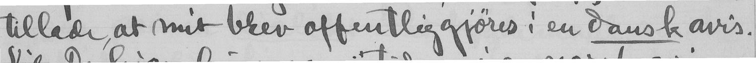tillade, at mit brev offentliggjøres i en dansk avis.
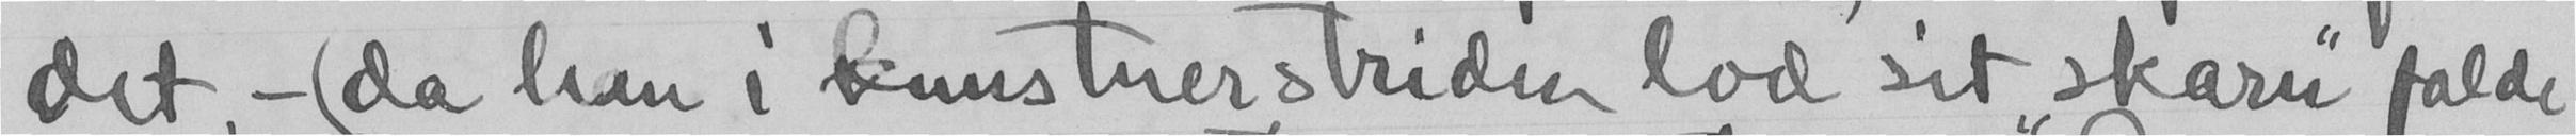det - (da han i kunstnerstriden lod sit "skarn" falde
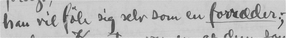han vil føle sig selv som en forræder, -
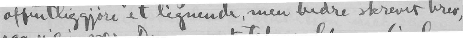offentliggjøre et lignende, men bedre skrevet brev,
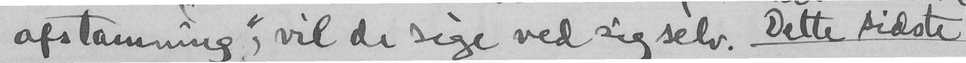afstanning", vil de sige ved sig selv. Dette sidste
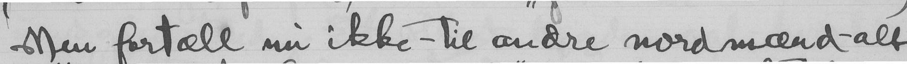Men fortæll nu ikke - til andre nordmænd alt
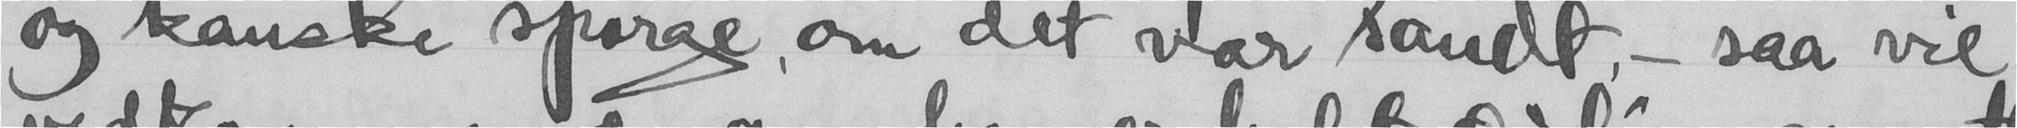og kanske spørge, om det var sandt - saa vil
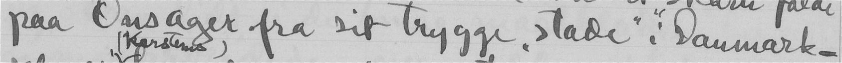paa Onsager fra sit trygge "stade" i Danmark-
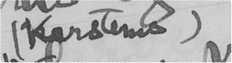(Karstens)
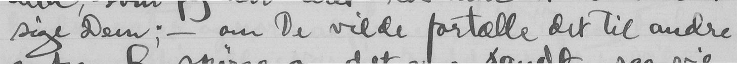sige Dem; - om De vilde fortælle det til andre
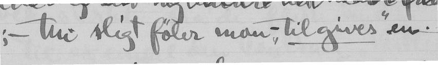; - thi sligt føler man, "tilgives" en
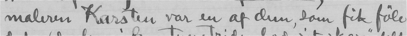maleren Karsten var en af dem som fik føle
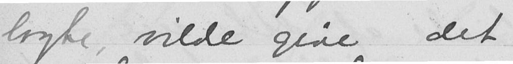lagte, vilde give det
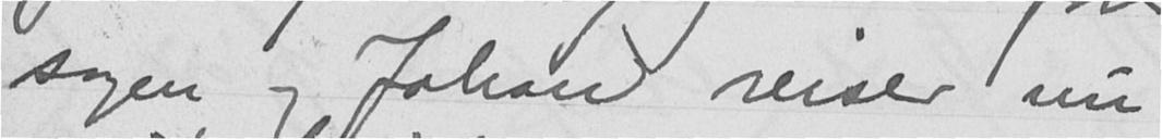sagen og Johan reiser nu
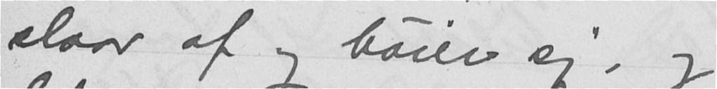slaar af og bøier sig, og
